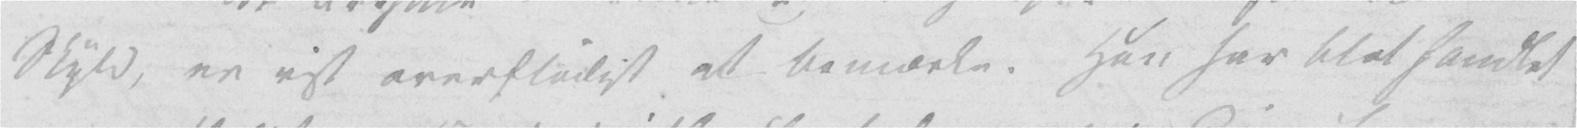Skyld, er vist overflødigt at bemærke. Han har blot handlet
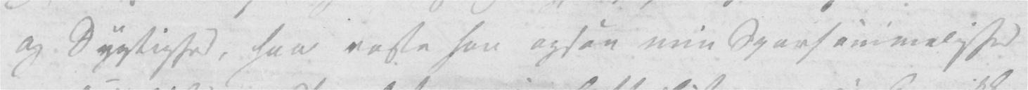og Dygtighed, saa roste han ogsaa min Sparsommelighed
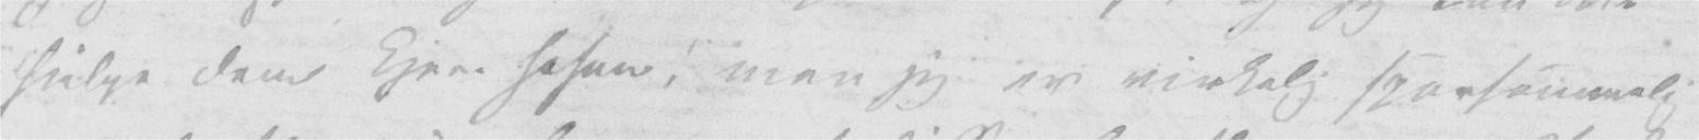hjelpe dem kjere Johan, men jeg er virkelig sparsommelig
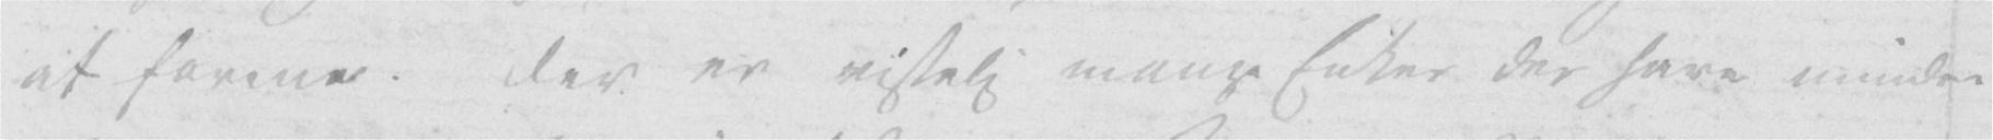at forene. Der er visselig mange Enker der have mindre
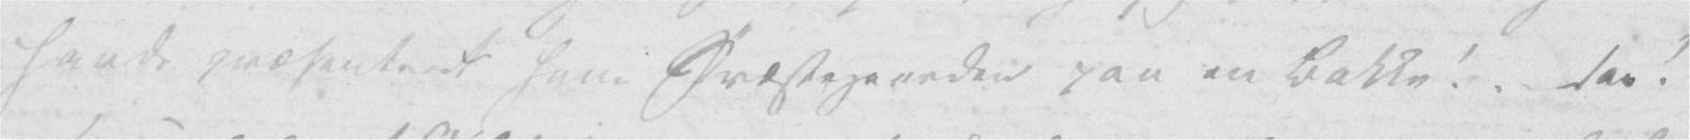havde præsenteret ham Præstegaarden paa en Bakke!.. saa?
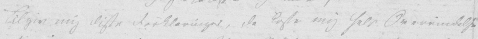Tilgiv mig disse Forklaringer, de koste mig selv Overvindelse
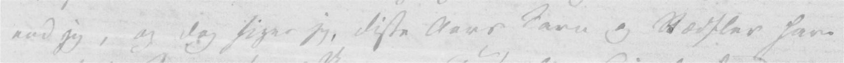end jeg, og dog siger jeg, disse Aars Savn og Rædsler have
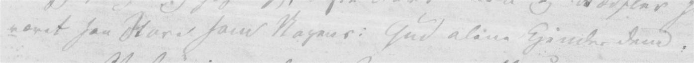været saa Store som Nogens: Gud alene kjender dem.
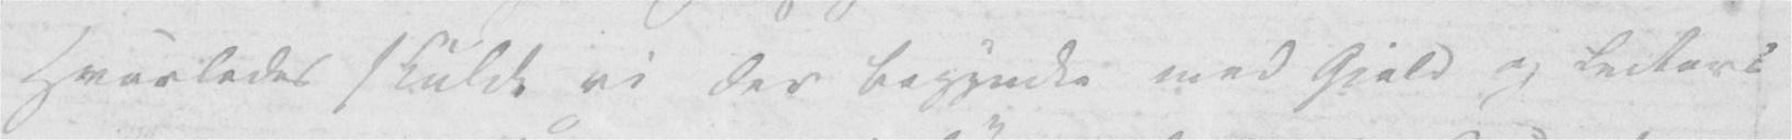Hvorledes skulde vi der begyndte med Gjeld og Lectors
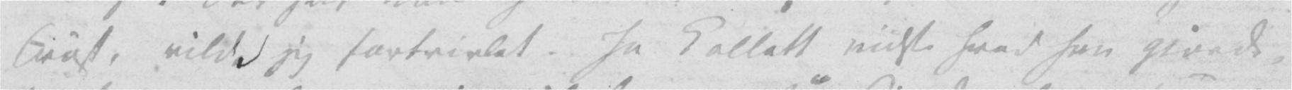Trøst, vilde jeg fortvivlet. Ja Collett vidste hvad han gjorde,
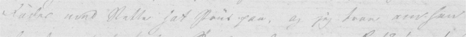Fader med Rette sat Priis paa, og jeg tror om han
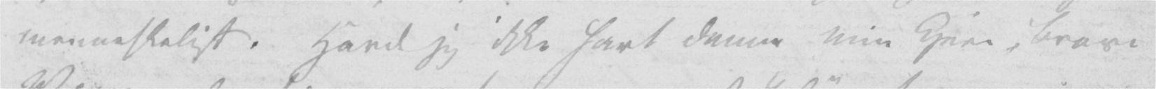menneskeligt. Havde jeg ikke havt denne min kjere, brave
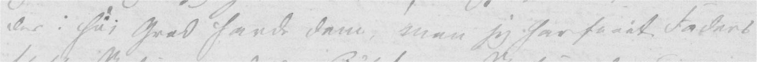der i høi Grad havde dem, men jeg har faaet Faders
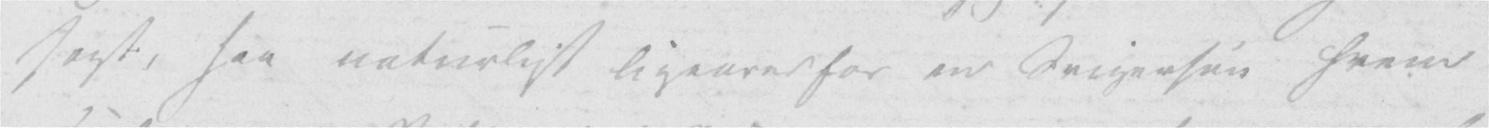sagt, saa naturligt ligeoverfor en Svigersøn hvem
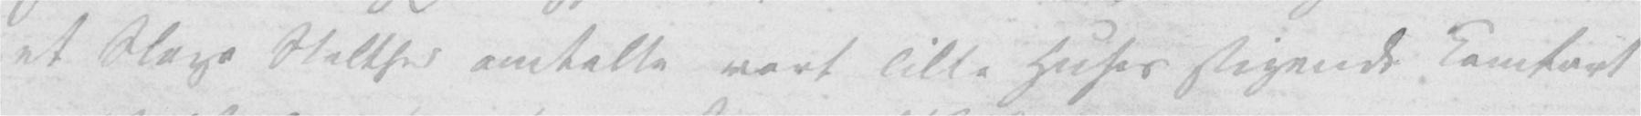et Slags Stolthed omtalte vort lille Huses stigende Komfort
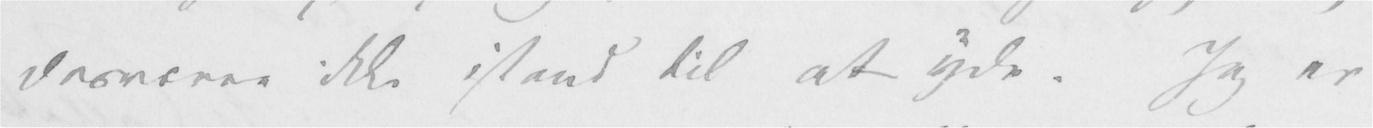desværre ikke istand til at yde. Jeg er
